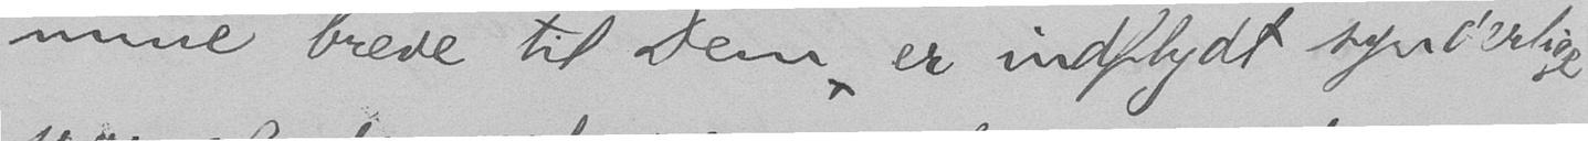mine breve til Dem, er indflydt synderlige
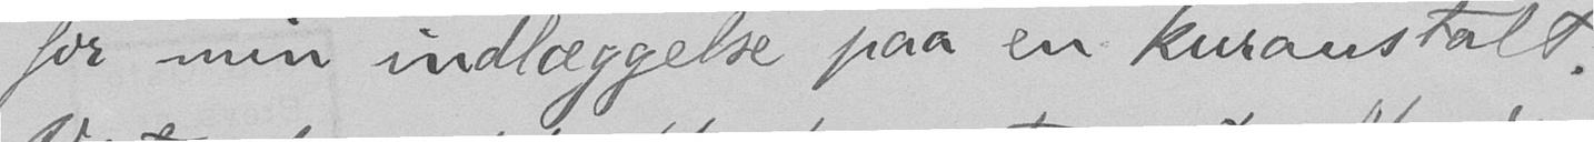for min indlæggelse paa en kuranstalt.
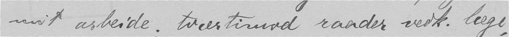mit arbeide; tværtimod raader vedk. læge,
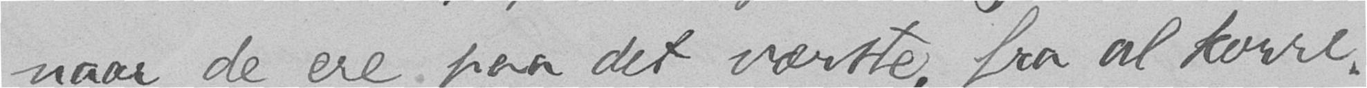naar de ere paa det værste, fra al korre-
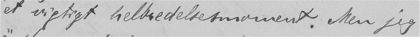et vigtigt helbredelsesmoment. Men jeg
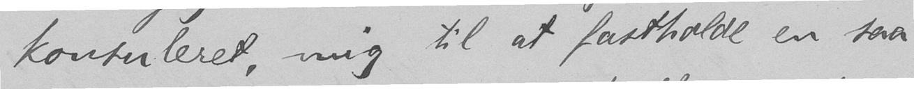konsuleret, mig til at fastholde en saa-
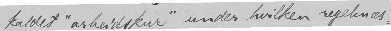kaldet "arbeidskur", under hvilken regelmæs-
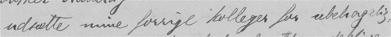udsætte mine forrige kolleger for ubehagelig-
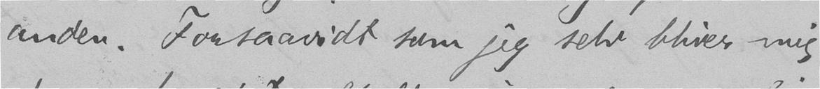anden. Forsaavidt som jeg selv bliver mig
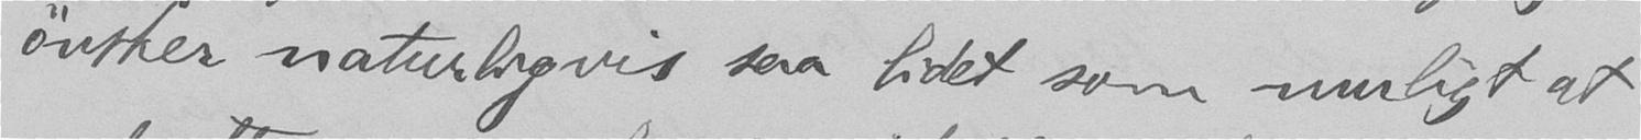ønsker naturligvis saa lidet som muligt at
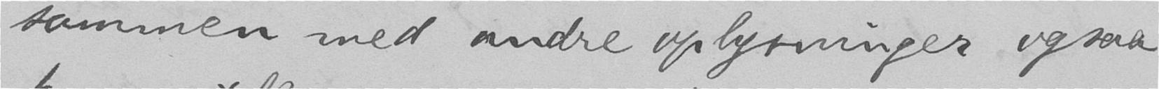sammen med andre oplysninger ogsaa
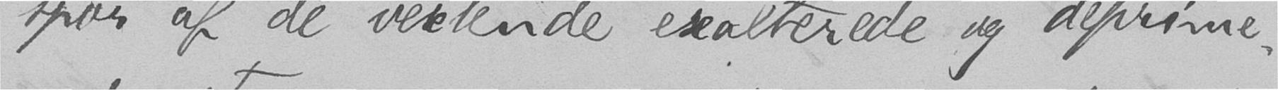spor af de vexlende exalterede og deprime-
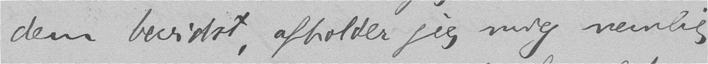dem bevidst, afholder jeg mig nemlig,
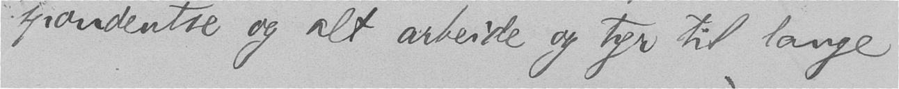spondentse og alt arbeide og tyr til lange
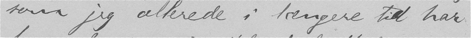som jeg allerede i længere tid har
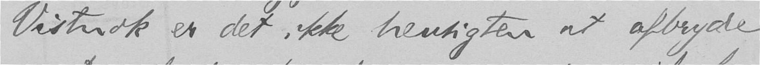Vistnok er det ikke hensigten at afbryde
